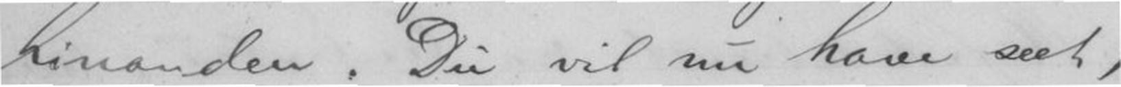hinanden. Du vil nu have seet,
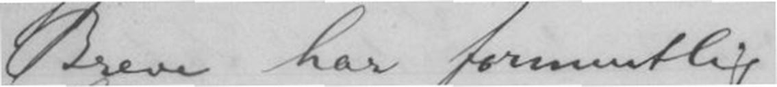Breve har formentlig
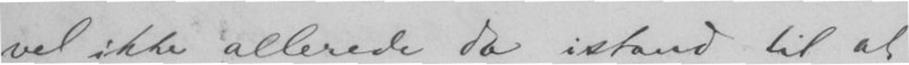vel ikke allerede da istand til at
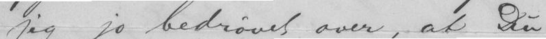jeg jo bedrøvet over, at Du
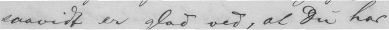saavidt er glad ved, at Du har
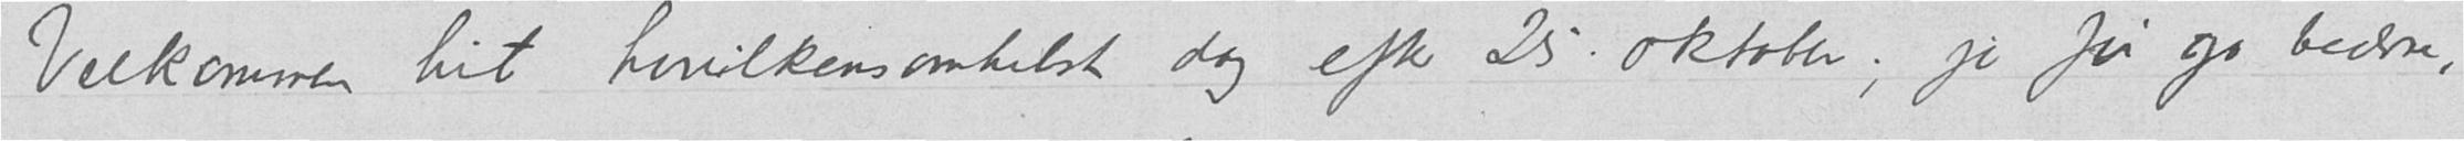Velkommen hit hvilkensomhelst dag efter 25. oktober; jo før jo bedrre,
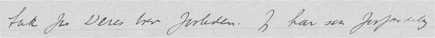tak for Deres brev forleden. Jeg har saa forfærdelig
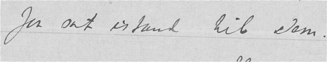faa sat istand til Dem.
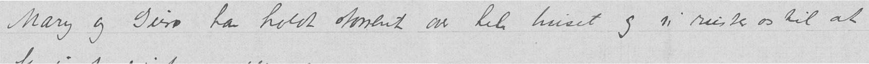Mary og Guro har holdt storrent over hele huset og vi ruster os til at
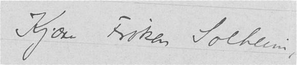Kjære Frøken Solheim,
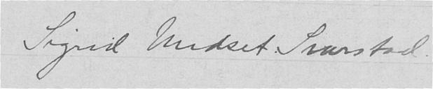Sigrid Undset Svarstad.
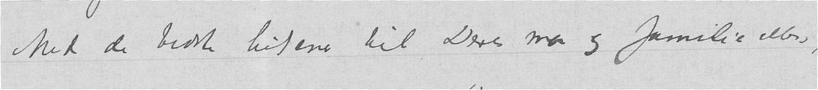Med de bedste hilsener til Deres mor og familie ellers,
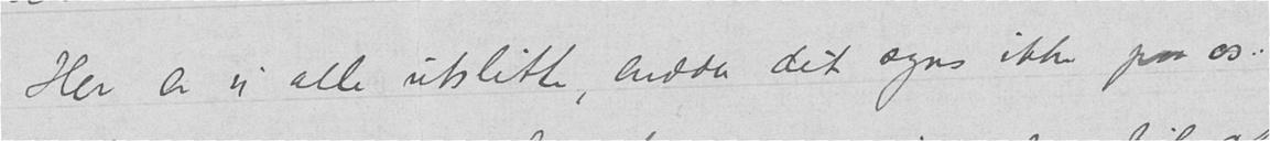Her er vi alle utslitte, endda det syns ikke paa os.
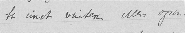ta imot vinteren ellers ogsaa.
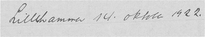Lillehammer 14. oktober 1922.
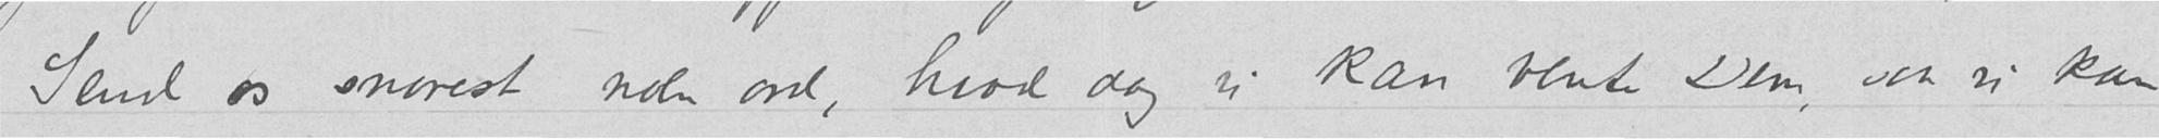Send os snarest noen ord, hvad dag vi kan vente Dem, saa vi kan
